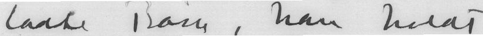ladte Børse, han holdt
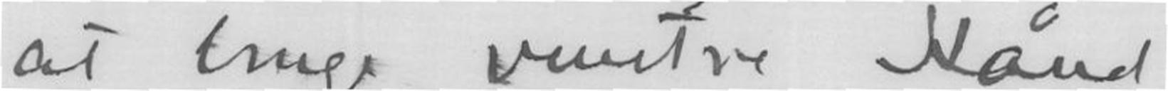at bruge venstre Hånd
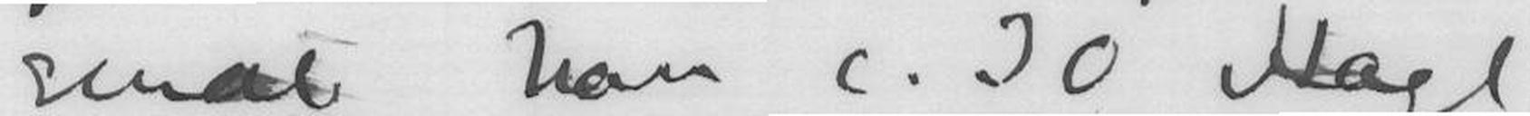sendt han c. 30 Hagl
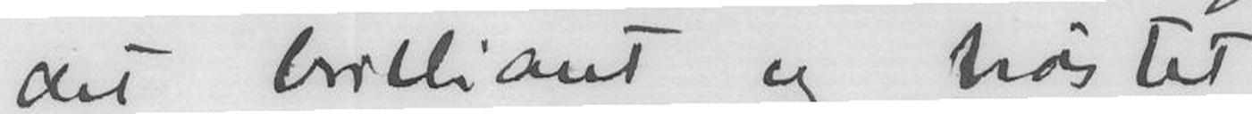det brilliant og trøstet
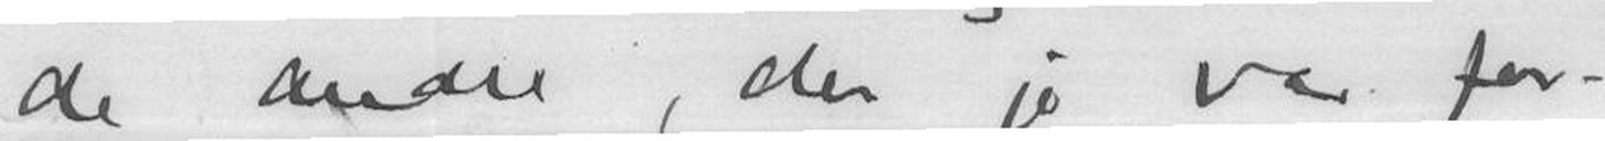de andre, der jo var for-
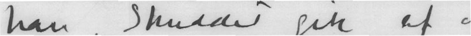han, skuddet gik af og
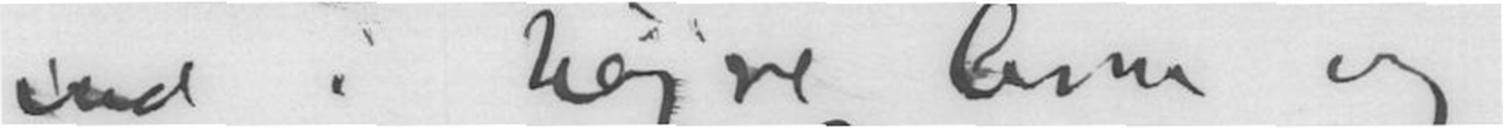ind i Højre Arm og
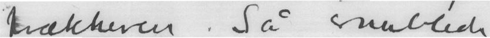trækkeren. Så snublede
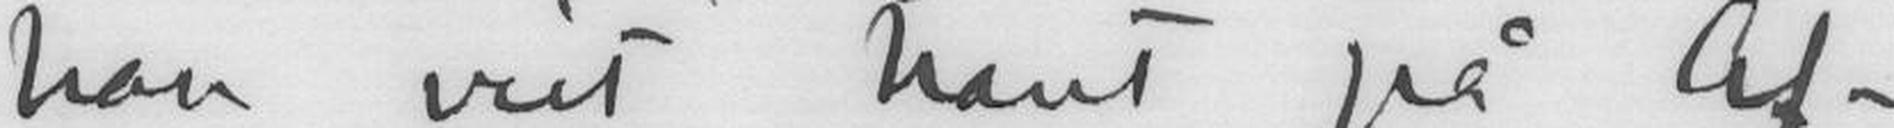han vist havt på Af-
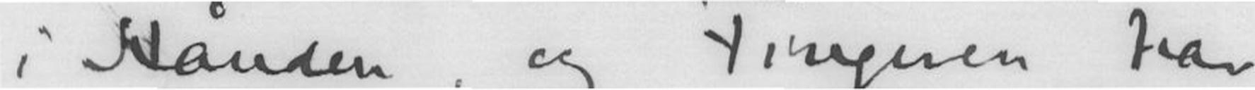i Hånden, og Fingeren har
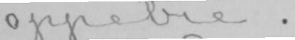oppebie.
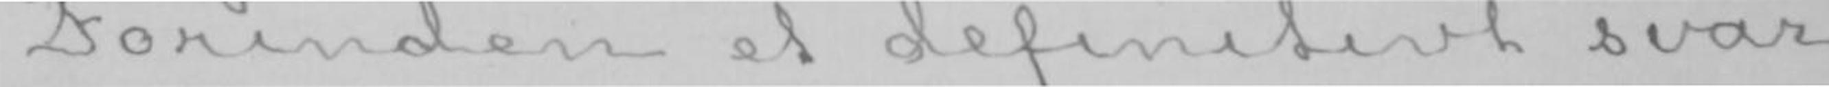Forinden et definitivt svar
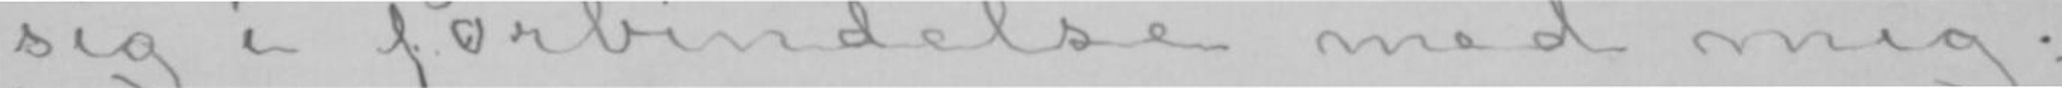sig i forbindelse med mig;
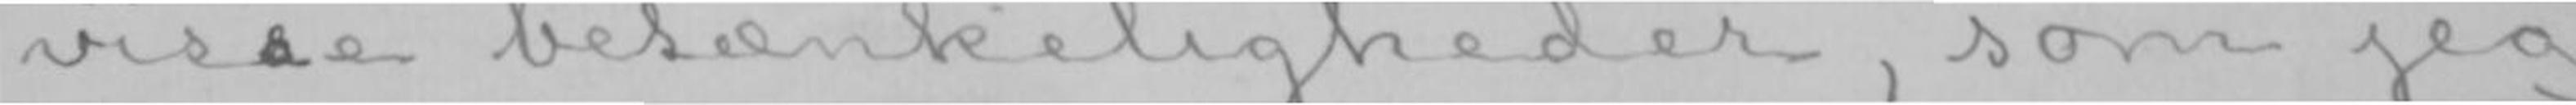visse betænkeligheder, som jeg
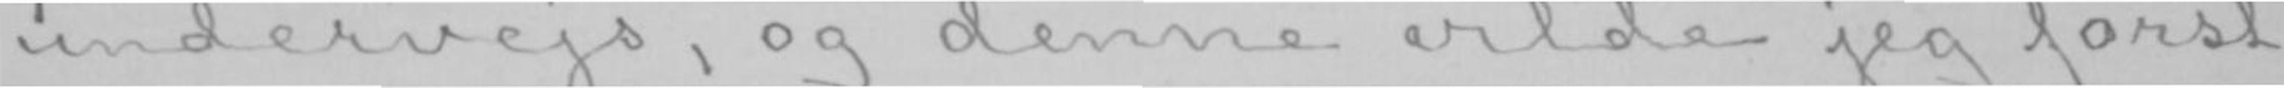undervejs, og denne vilde jeg først
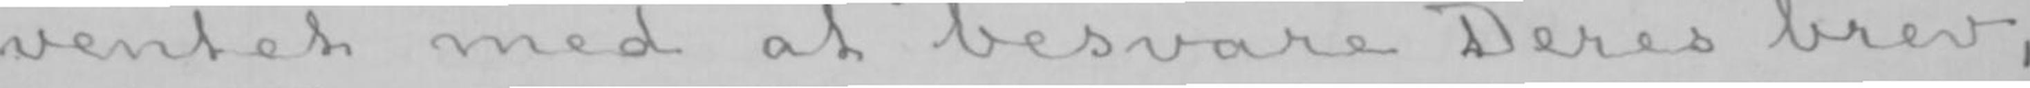ventet med at besvare Deres brev,
